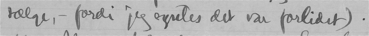sælge, - fordi jeg syntes det var forlidet).
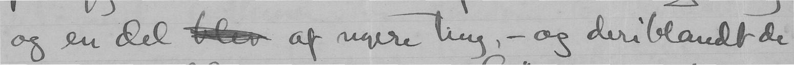og en del  af nyere ting, - og deriblandt de
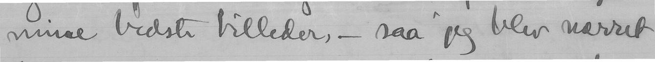mine bedste billeder, - saa jeg blev narret
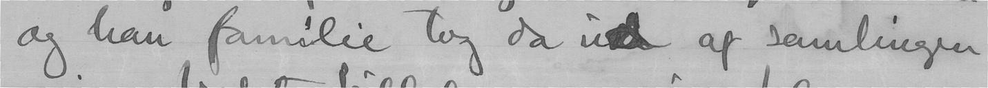og han familie tog da ud af samlingen
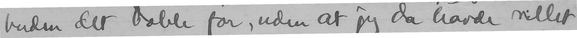buden det doble for, uden at jeg da havde villet
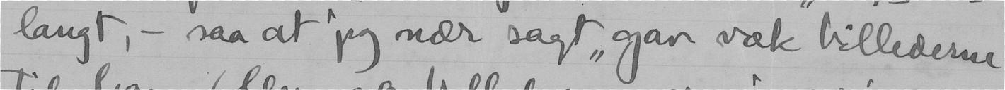langt, - saa at jeg nær sagt "gav væk" billederne
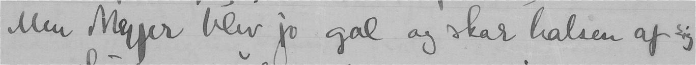Men Meyer blev jo gal og skar halsen af sig
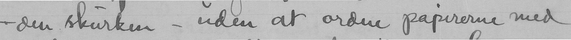- den skurken - uden at ordne papirerne med
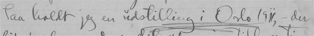Saa holdt jeg en udstilling i Oslo 1911, - der
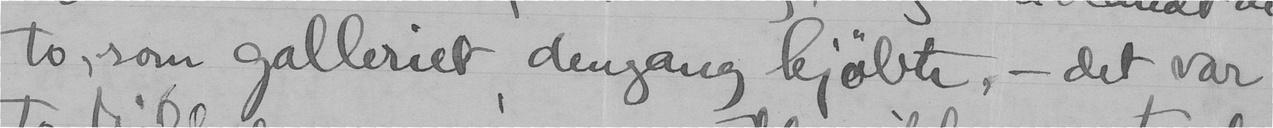to, som galleriet dengang kjøbte, - det var
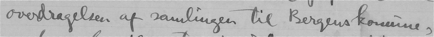overdragelsen af samlingen til Bergens komune,
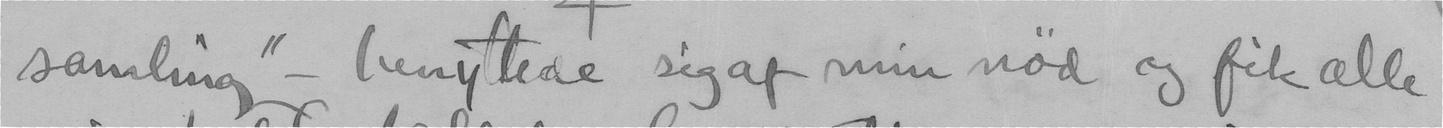samling" - benyttede sig af min nød og fik alle
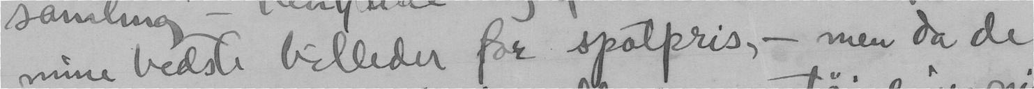mine bedste billeder for spotpris, - men da de
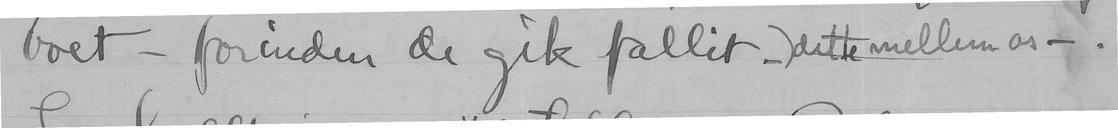boet - forinden de gik fallit -) dette mellem os -.
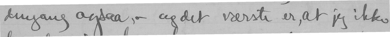dengang ogsaa, - og det værste er, at jeg ikke
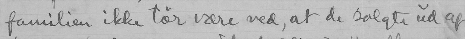familien ikke tør være ved, at de solgte ud af
